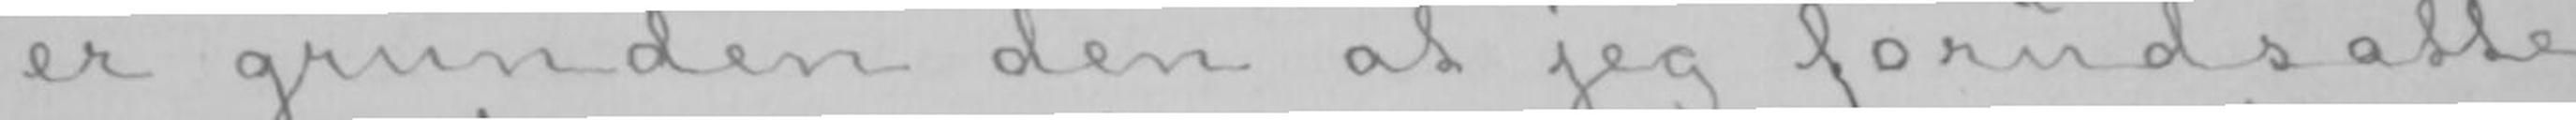er grunden den at jeg forudsatte
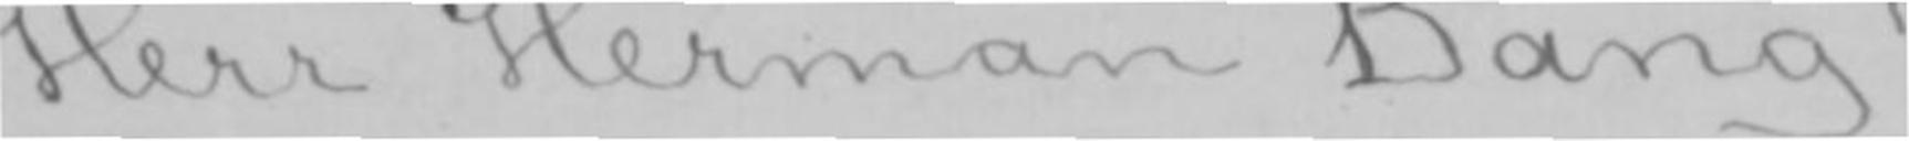Herr Herman Bang!
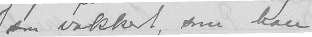saa vakkert, som han
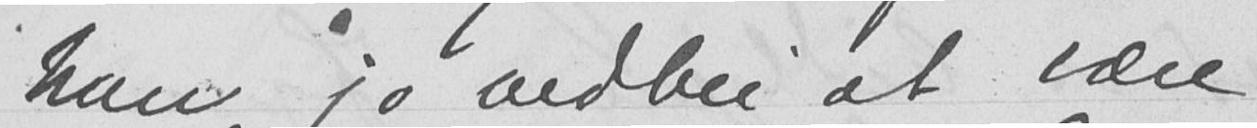han jo vedbli at være
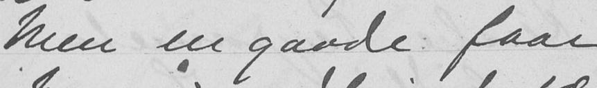Men en gaade faar
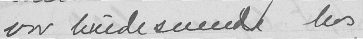var brudesvende hos
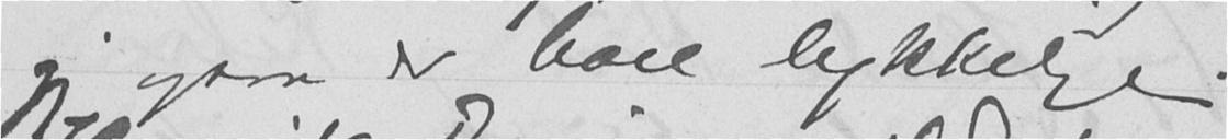jeg ogsaa er bare lykkelige.
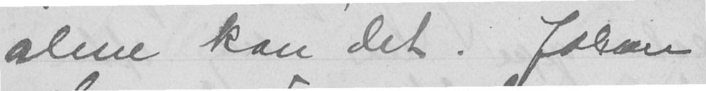alene kan det. Johan
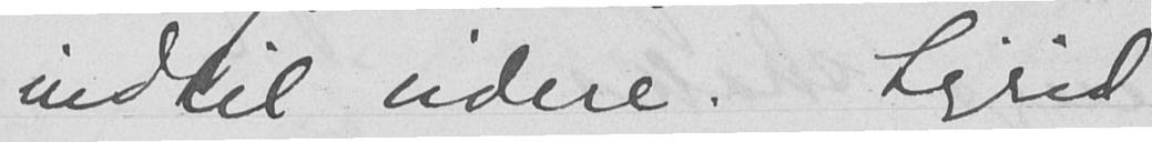indtil videre. Sigrid
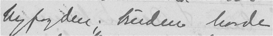byfogden; bruden havde
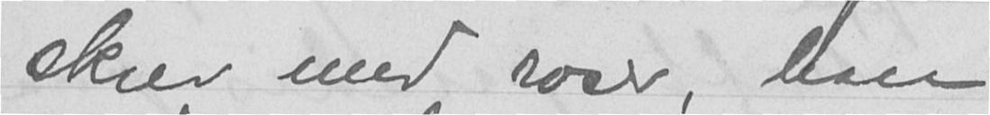skrev med roser, han
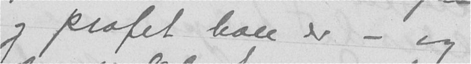og profet han er - og
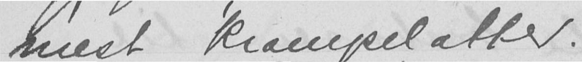mest krampelatter.
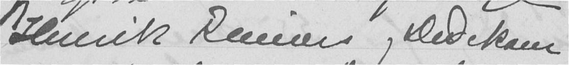Henrik Reimers og Dedekam
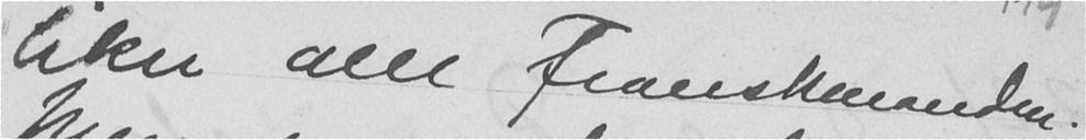liker alle Franskmanden.
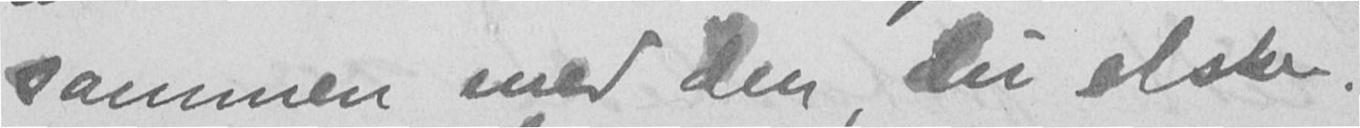sammen med den, du elsker.
